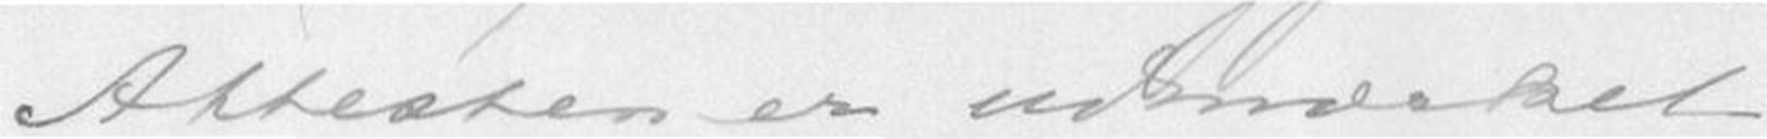Attesten er udmerket,
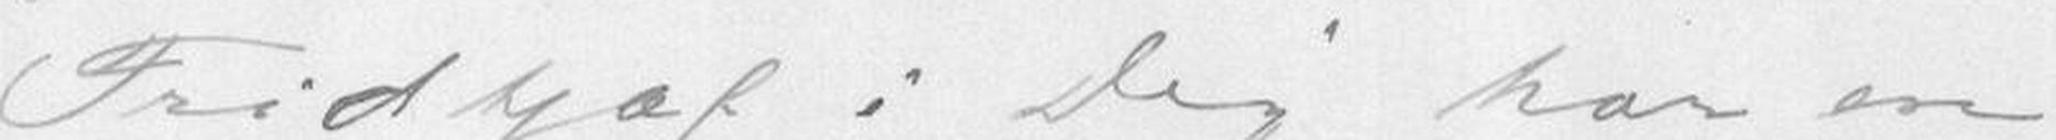Fridtjof i Dig har en
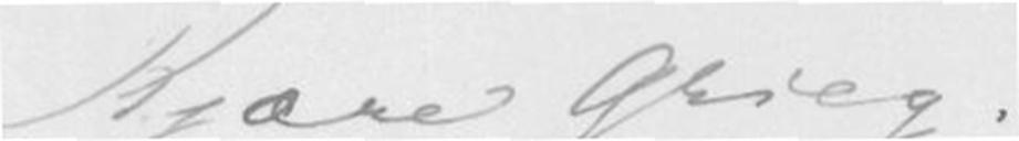Kjære Grieg.
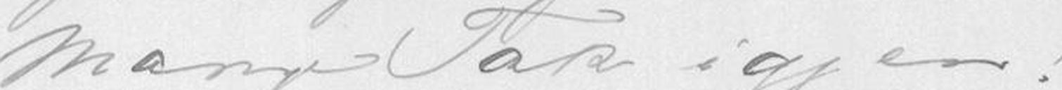Mange Tak igjen!
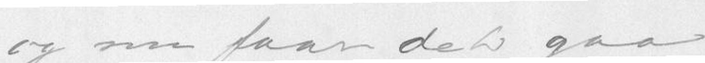og nu faar det gaa
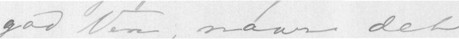god Ven, naar det
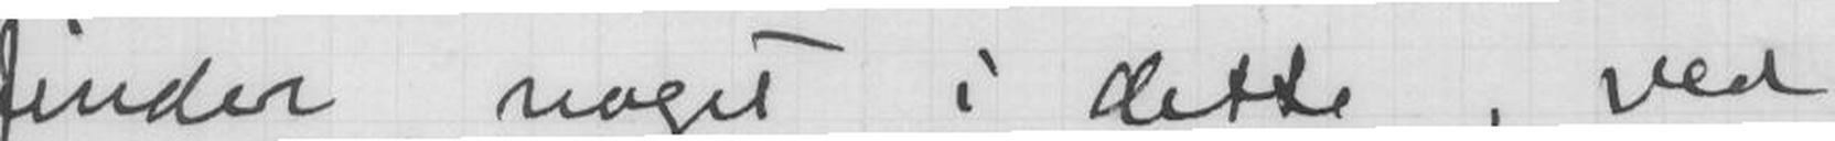finder noget i dette,
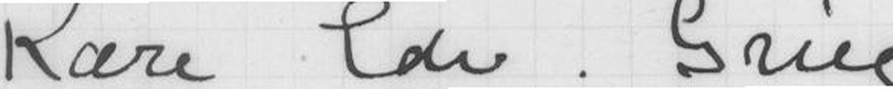Kære Edv. Grieg
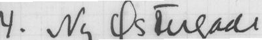4. Ny Østergade.
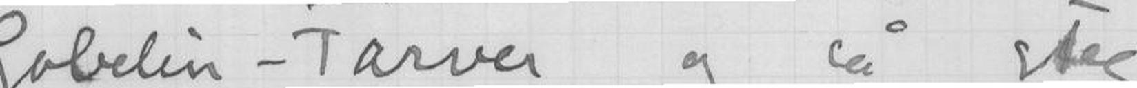Gobelin-Farver og så steg
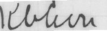Kbhvn.
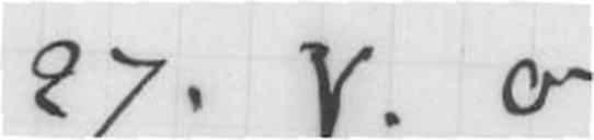27.V.07
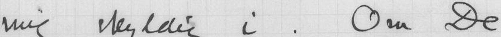mig skyldig i. Om De
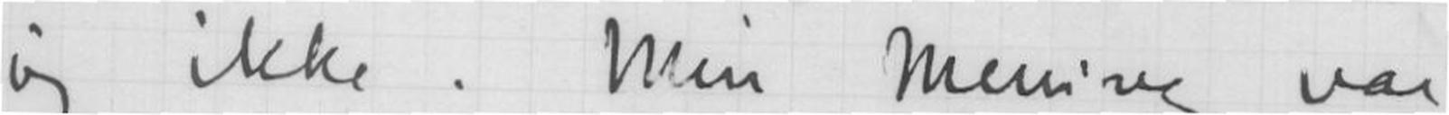ved jeg ikke. Min Mening var
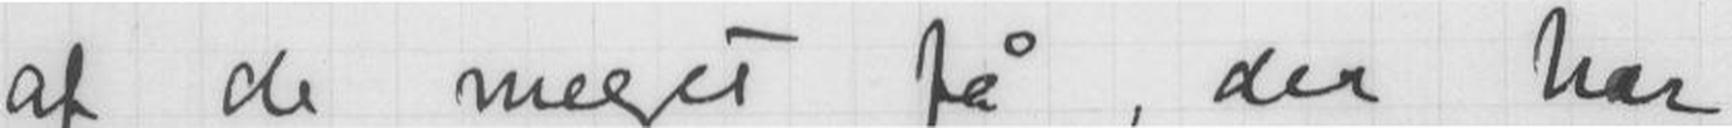af de meget få, der har
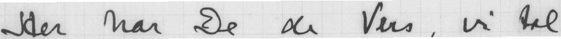Her har De de Vers, vi tal
